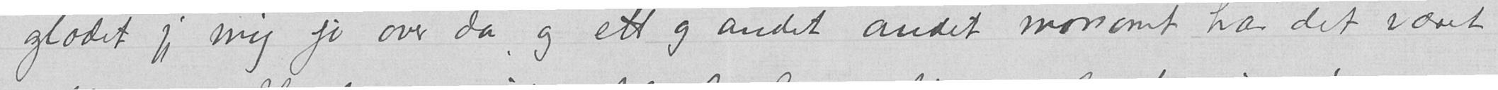glædet jeg mig jo over da, og ett og andet andet morsomt har det været
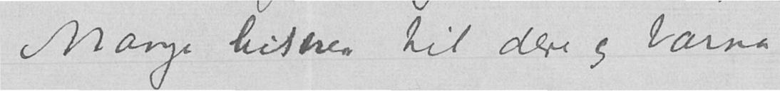Mange hilsener til dere og barna
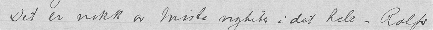Det er nokk av triste nyheter i det hele - Rolfs
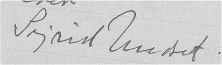Sigrid Undset.
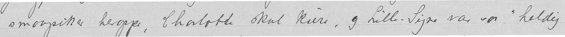smaapiker heroppe, Charlotte skal kure, og Lille-Signe var saa "heldig"
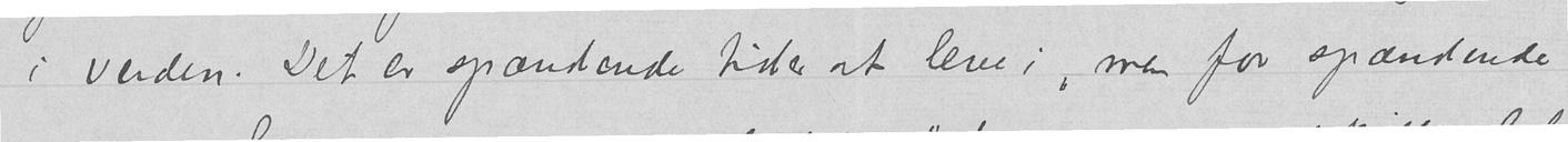i verden. Det er spændende tider at leve i, men for spændende
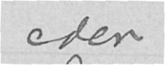eders
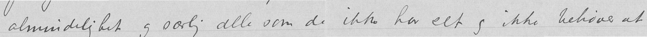almindelighet og særlig alle som de ikke har set og ikke behøver at
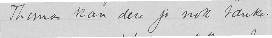Thomas kan dere jo nok tænke.
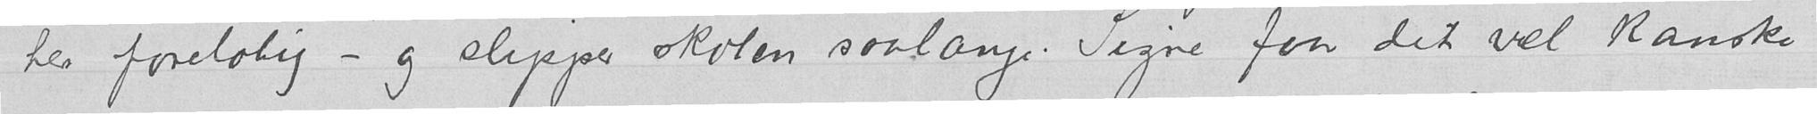her foreløbig - og slipper skolen saalænge. Signe faar det vel kanske
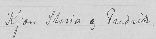Kjære Stina og Fredrik,
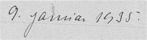9. januar 1935.
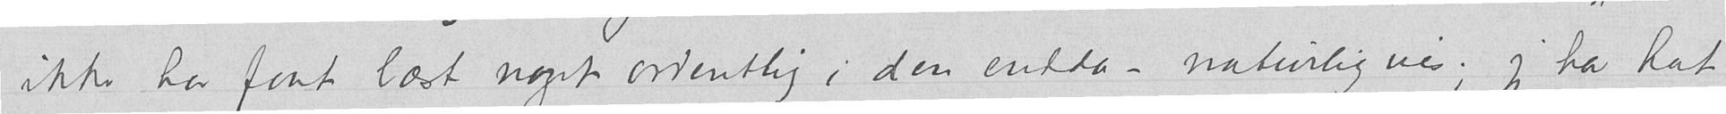ikke har faat læst noget ordentlig i den endda – naturligvis; jeg har hat
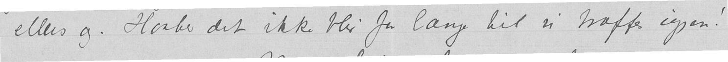ellers og. Haaber det ikke blir for længe til vi træffes igjen!
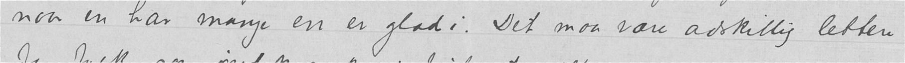naar en har mange en er glad i. Det maa være adskillig lettere
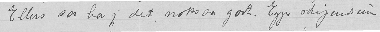Ellers saa har jeg det noksaa godt. Egges stipendium
